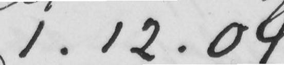1.12.04
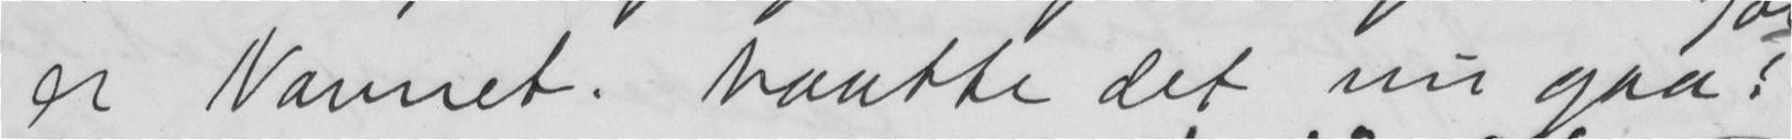er Navnet. Maatte det nu gaa!
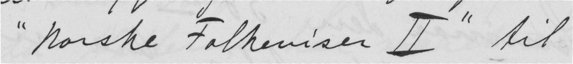"Norske Folkeviser II" til
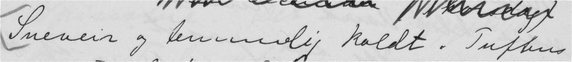Sneveir og temmerlig koldt. Tuftens
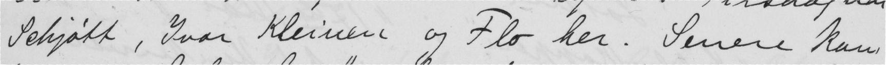Schjøtt, Ivar Kleiven og Flo her. Senere kom
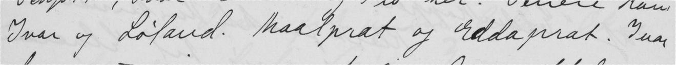Ivar og Løland. Maalprat og Eddaprat. Ivar
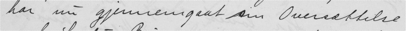har nu gjennemgaat sin Oversættelse
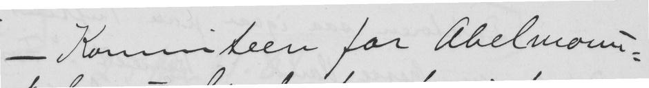- Kommiteen for Abelmonu-
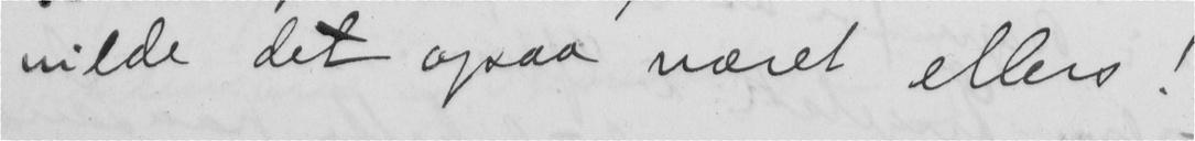vilde det ogsaa været ellers!
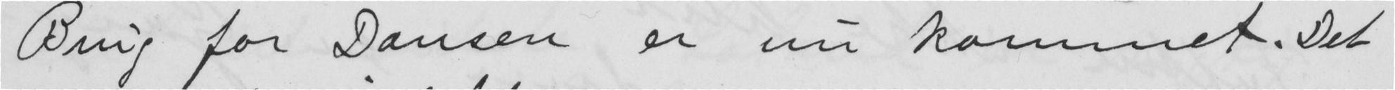Brug for Dansen er nu kommet. Det
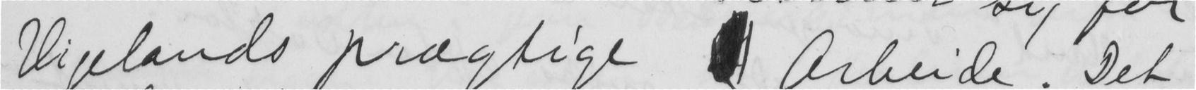Vigelands prægtige  Arbeide. Det
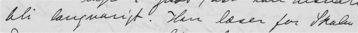bli langvarigt. Han læser for Skolen
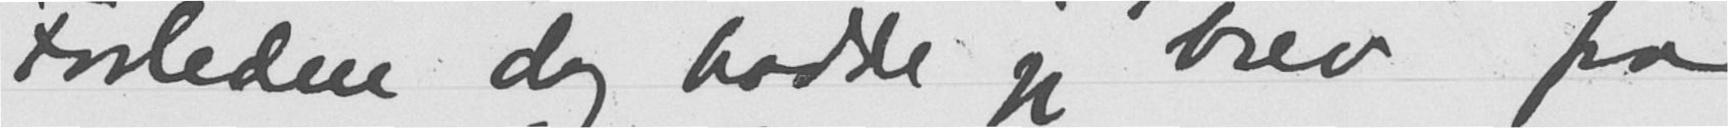Forleden dag hadde jeg brev fra
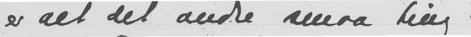er alt det andre smaa ting.
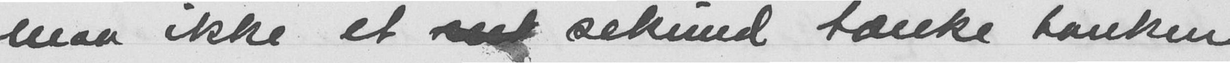maa ikke et sekund tænke tanken
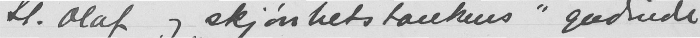St.Olaf og „skjønhetstankens” gudinde
